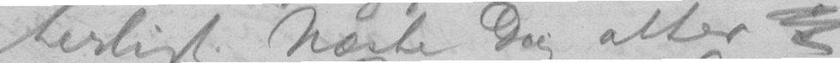herligt. Næste Dag atter
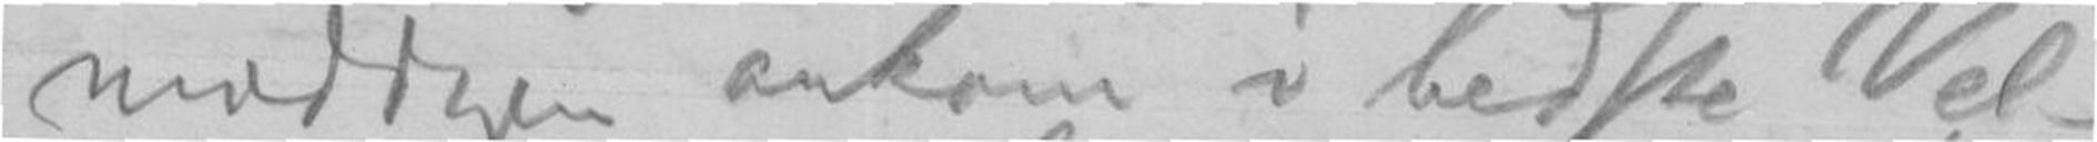middagen amkom i bedste Vel-
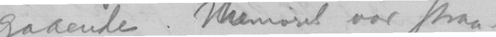gaaende. Humøret var straa-
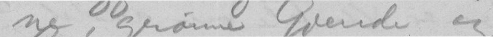ne, grønne Gjende og
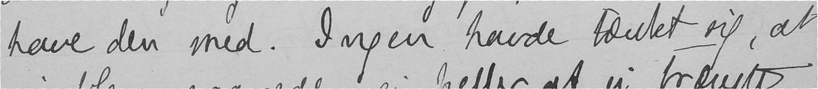have den med. Ingen havde tænkt sig, at
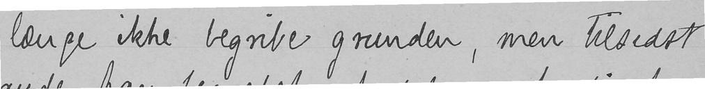længe ikke begribe grunden, men tilsidst
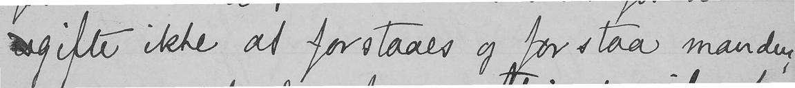ugifte ikke at forstaaes og forstaa manden,
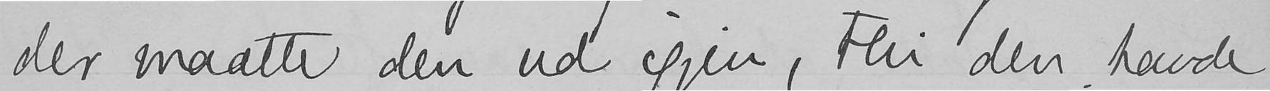der maatte den ud igjen, thi den havde
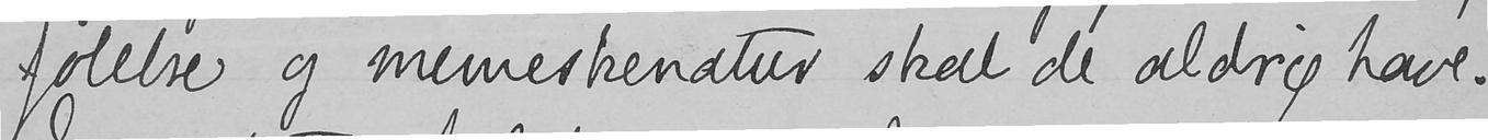følelse og menneskenatur skal de aldrig have.
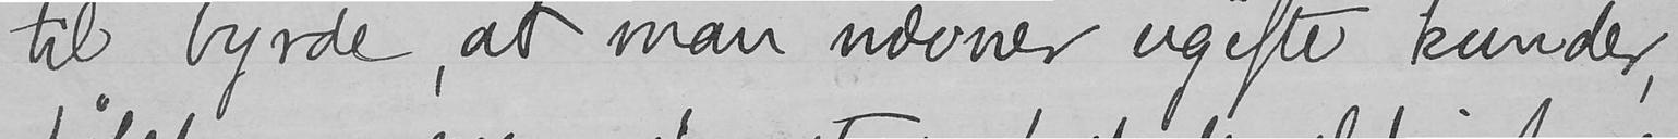til byrde, at man nævner ugifte kvinder,
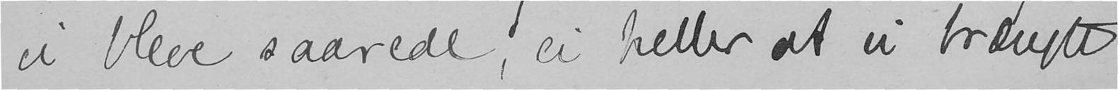vi bleve saarede, ei heller at vi trængte
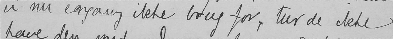vi nu engang ikke brug for, turde ikke
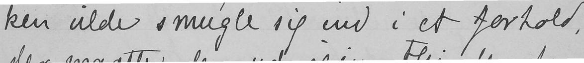ken vilde smugle sig ind i et forhold,
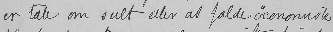er tale om sult eller at falde øconomisk
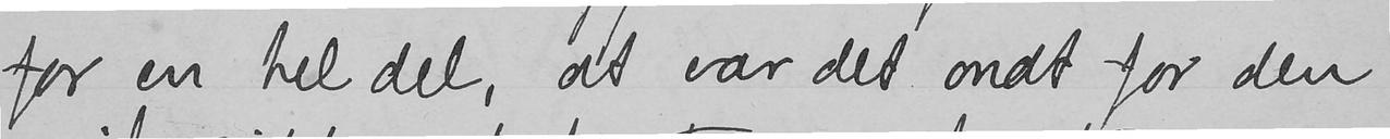for en hel del, at var det ondt for den
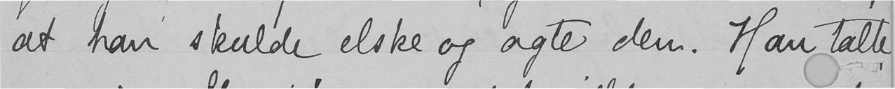at han skulde elske og agte dem. Han talte,
